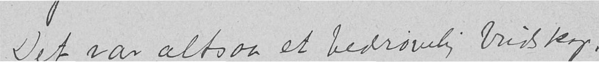Det var altsaa et bedrøvelig budskap,
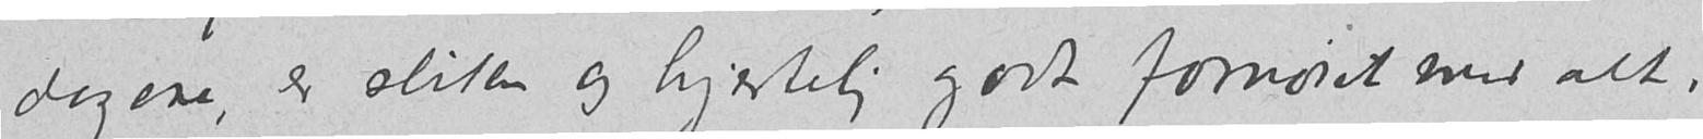dagene, er sliten og hjertelig godt fornøiet med alt,
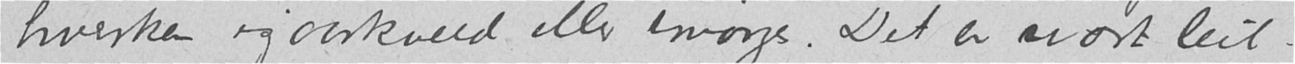hverken igaarkveld eller imorges. Det er svært leit
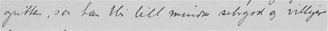gutten, saa han blir litt mindre selvgod og villigere
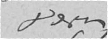Deres
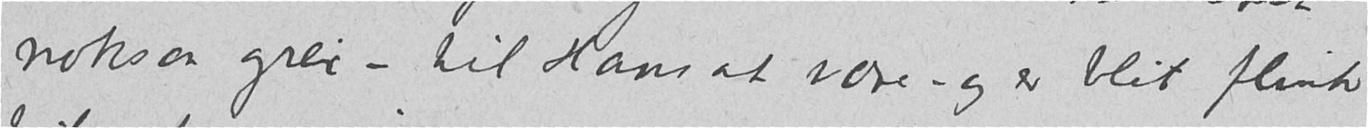noksaa grei – til Hans at være – og er blit flink
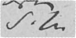S.U.
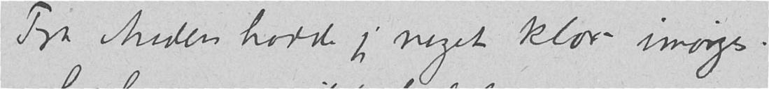Fra Anders hadde jeg noget klor imorges.
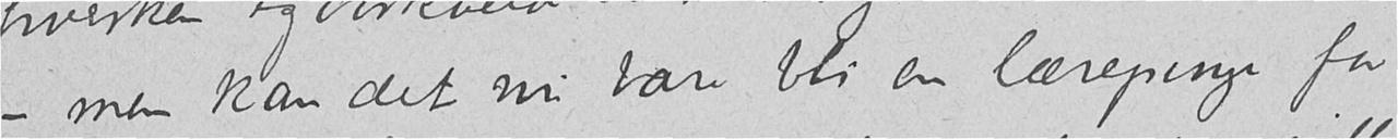– men kan det nu bare bli en lærepenge for
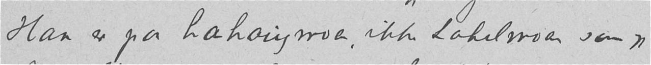Han er paa Lahaugmoen, ikke Lahelmoen som jeg
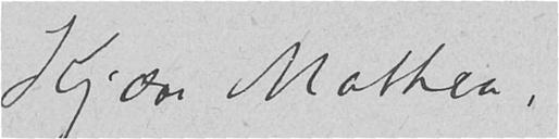Kjære Mathea,
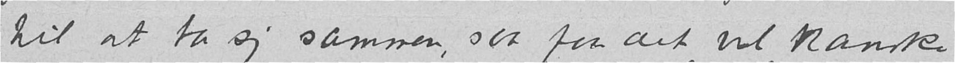til at ta sig sammen, saa faar det vel kanske
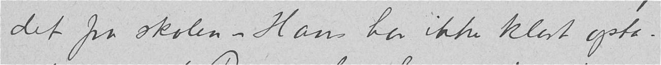det fra skolen – Hans har ikke klart opta-
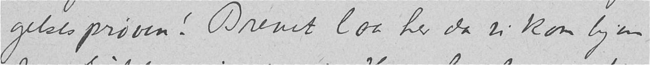gelsesprøven! Brevet laa her da vi kom hjem
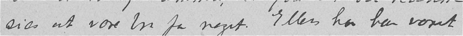sies at være bra for noget. Ellers har han været
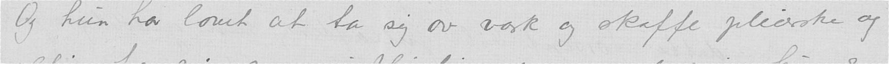Og hun har lovet at ta sig av vask og skaffe pleierske og
